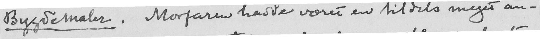Bygdemaler. Morfaren havde været en tildels meget an-
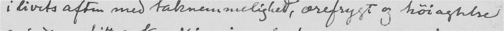i livets aften med taknemmelighet, ærefrygt og høiagtelse
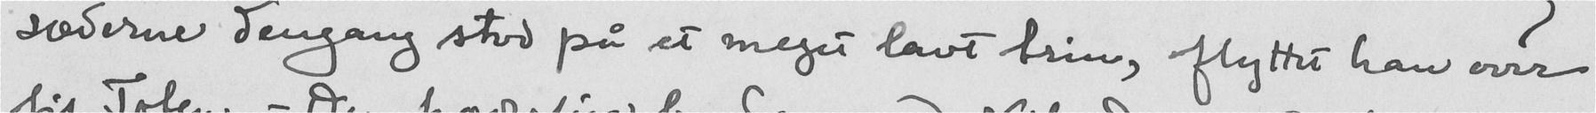sæderne dengang stod på et meget lavt trin, flyttet han over
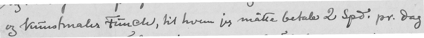og kunstmaler Funch, til hvem jeg måtte betale 2 spd. pr. dag
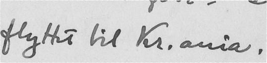flyttet til Kr.ania.
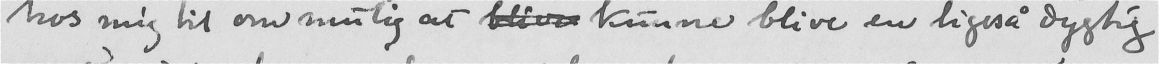hos mig til om mulig at blive kunne blive en ligeså dygtig
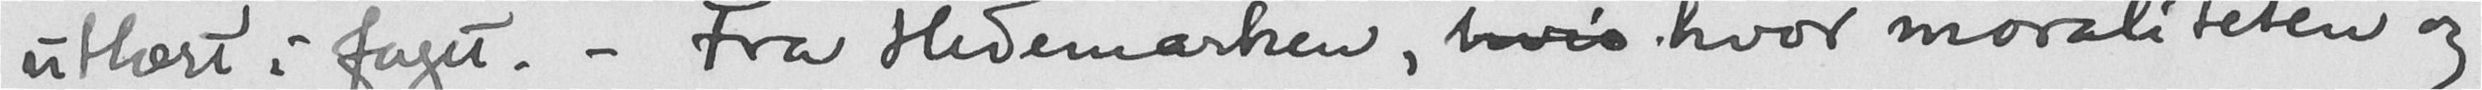utlært i faget. - Fra Hedemarken, hvis hvor moraliteten og
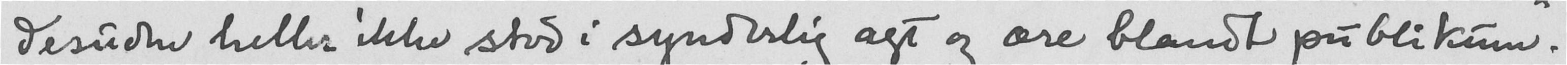desuden heller ikke stod i synderlig agt og ære blandt publikum."
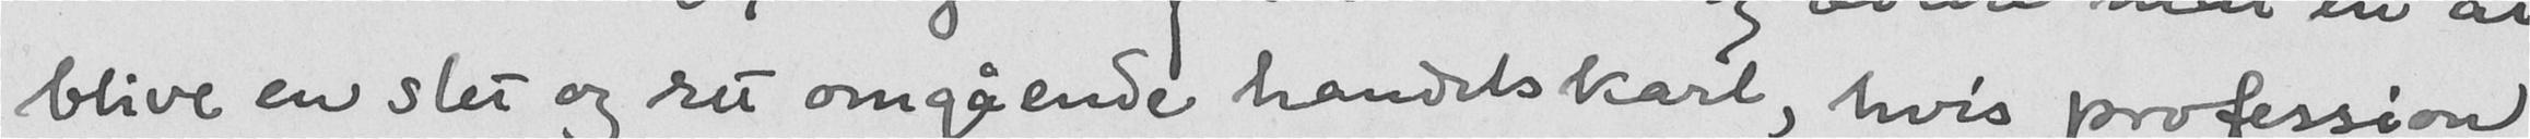blive en slet og ret omgående handelskarl, hvis profession
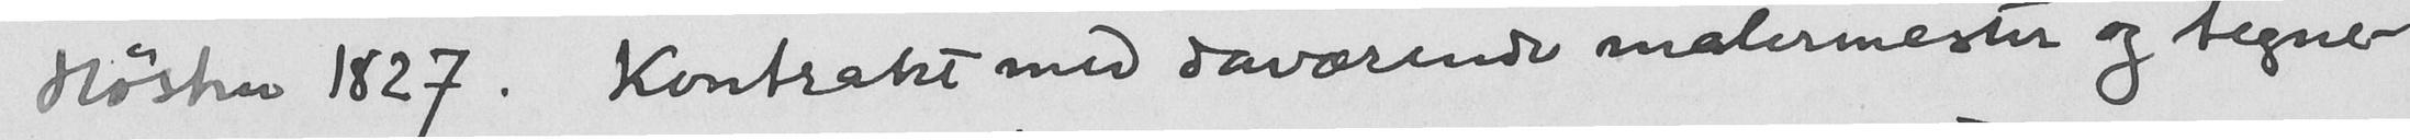Høsten 1827. Kontrakt med daværende malermester og tegne-
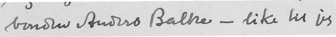bonden Anders Balke - like til jeg
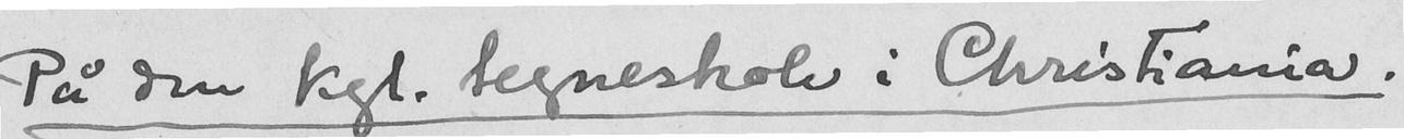På den kgl. tegnestole i Christiania.
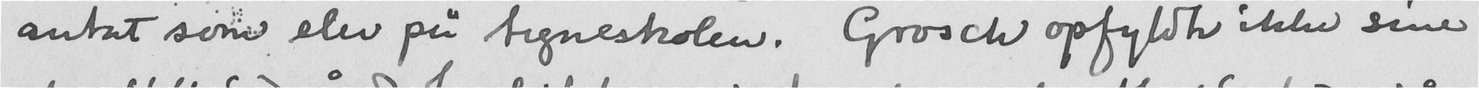antat som elev på tegneskolen. Grosch opfyldte ikke sine
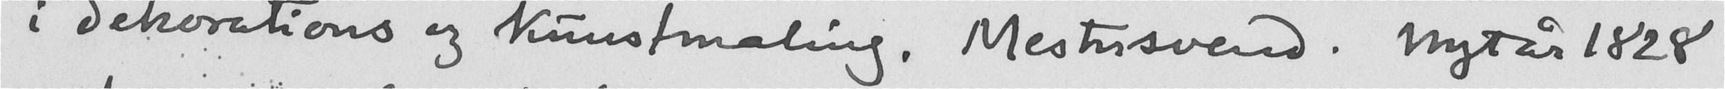i dekorations og kunstmaling. Mestersvend. Nytår 1828
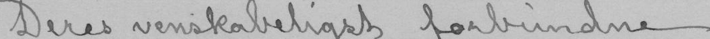Deres venskabeligst forbundne
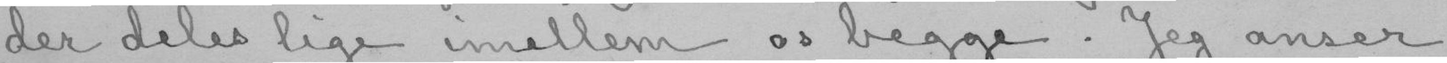der deles lige imellem os begge. Jeg anser
